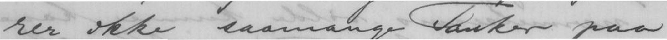rer ikke saamange Tanker paa
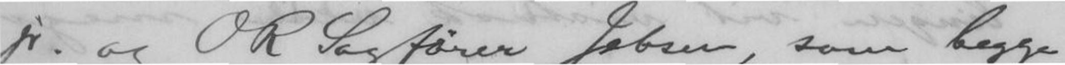jr. og ORSagfører Jebsen, som begge
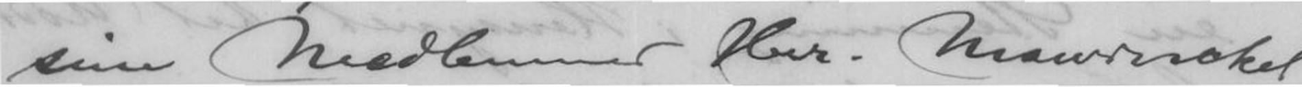sine Medlemmer Hrr. Mowinckel
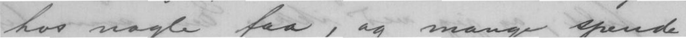hos nogle faa, og mange spende-
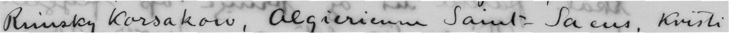Rimsky Korsakow, Algierienne Saint-Saeus, Kristi
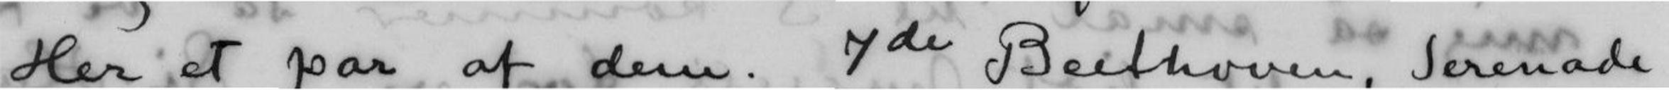Her et par af dem. 7de Beethoven, Serenade
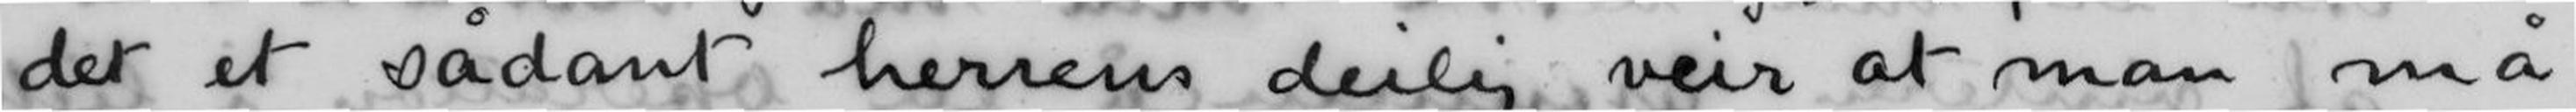det et sådant herrens deilig veir at man må
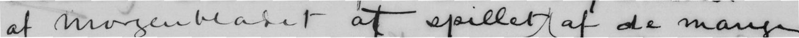af Morgenbladet at spillet af de mange
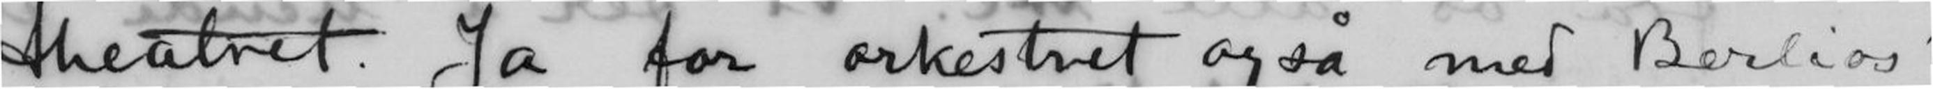theatret. Ja for orkestret også med Berlios'
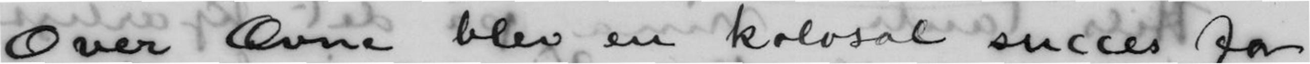Over Ævne blev en kolosal succes for
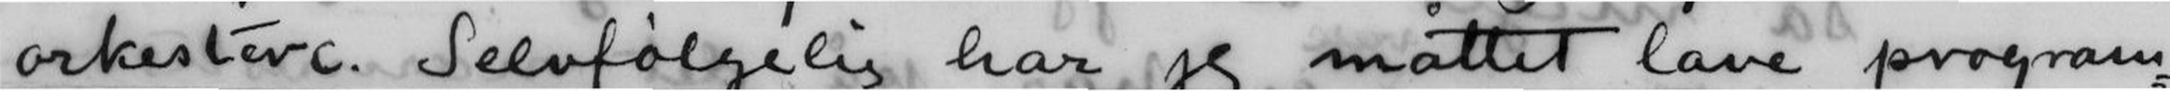orkester. Selvfølgelig har jeg måttet lave program-
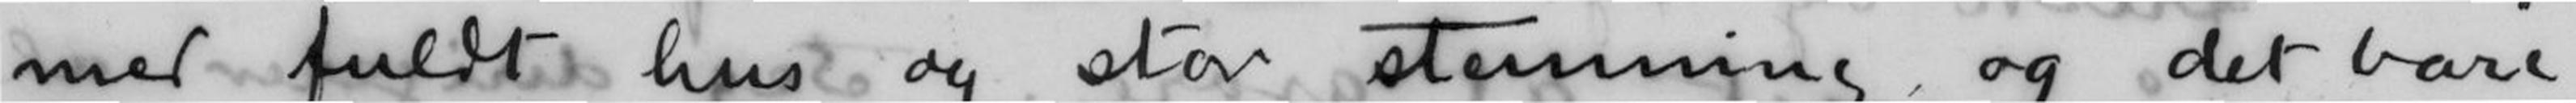med fuldt hus og stor stemning, og det bare
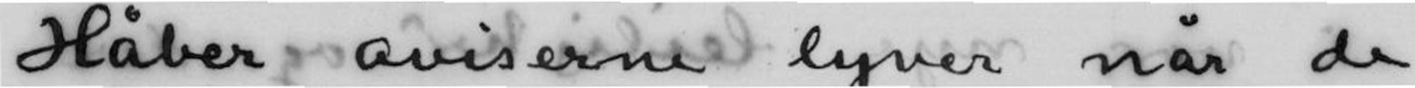Håber aviserne lyver når de
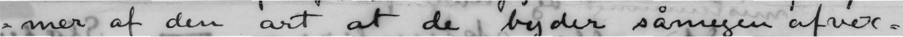mer af den art at de byder såmegen afvex-
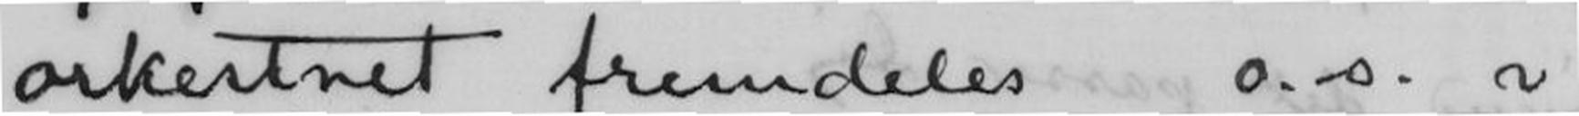orkestret fremdeles o.s.v.
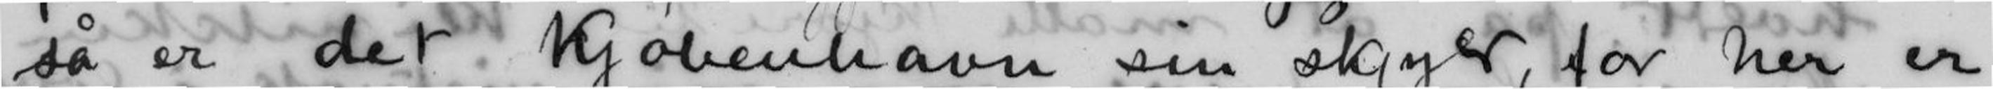så er det Kjøbenhavn sin skjyld, for her er
